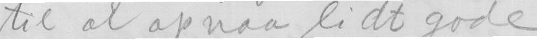til al opnaa lidt gode
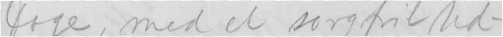Dage, med at sorgfrit Ud-
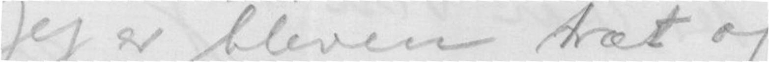Jeg er bleven træt og
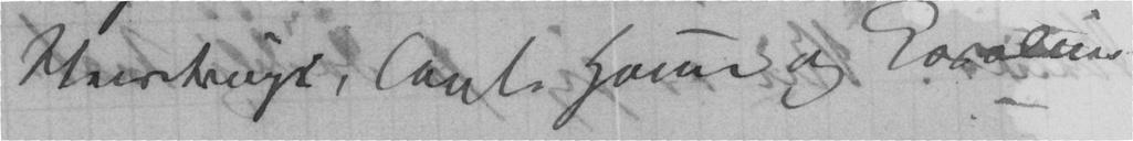Stenstrups, Tante Hanne og Caroline
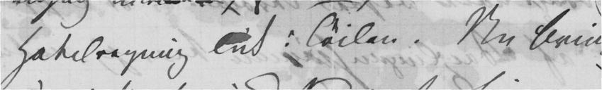Hotelregning lidt i Tøilen. Nu bringer
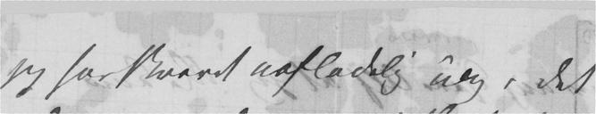jeg har skrevet uafladelig etc, det
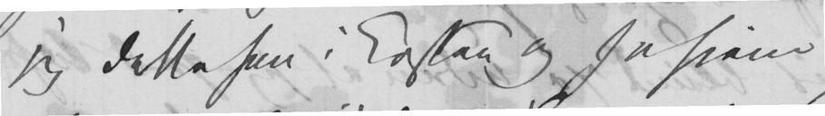jeg dette hen i Kassen og sa hjem
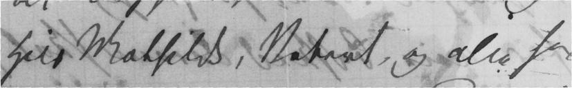Hils Mathilde, Robert, og alle hos
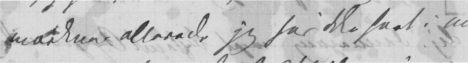mørkner allerede jeg har ikke havt i min
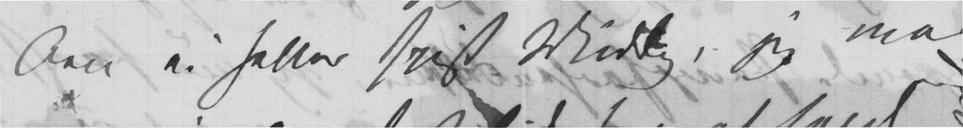Ovn ei heller spist Middag, jeg ma
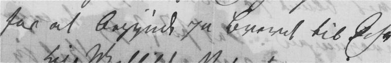for at begynde pa Brevet til Schwe.
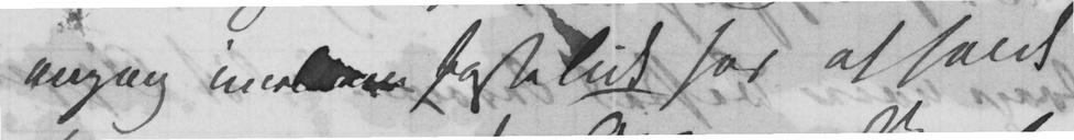engang imellem faste lidt for at holde
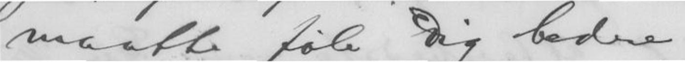maatte føle Dig bedre,
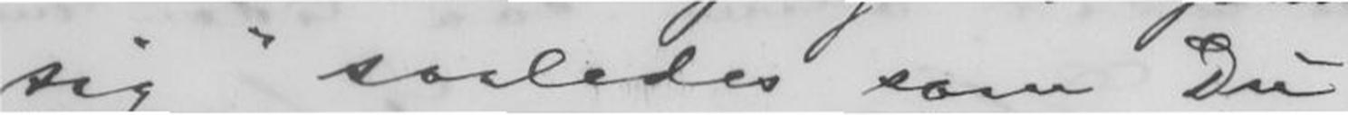sig" saaledes som Du
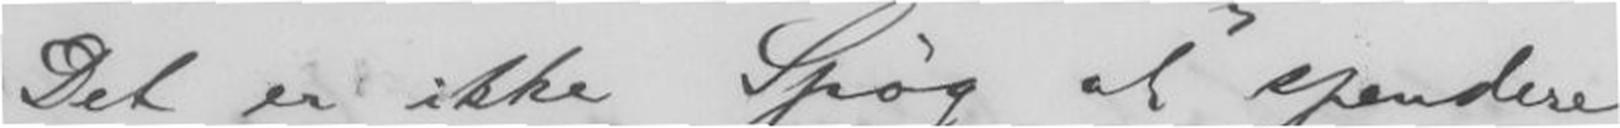Det er ikke Spøg at "spendere
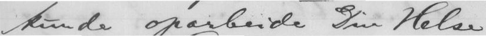kunde oparbeide Din Helse
